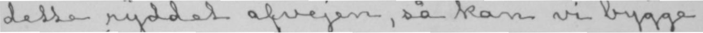dette ryddet afvejen, så kan vi bygge.
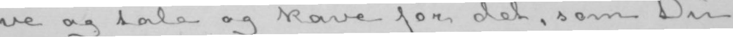ve og tale og kave for det, som Du
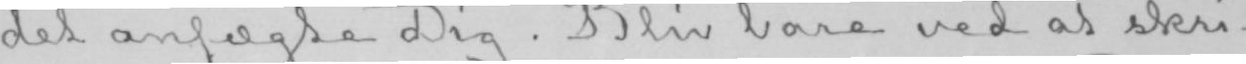det anfægte Dig. Bliv bare ved at skri-
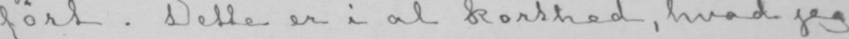ført. Dette er i al korthed, hvad jeg
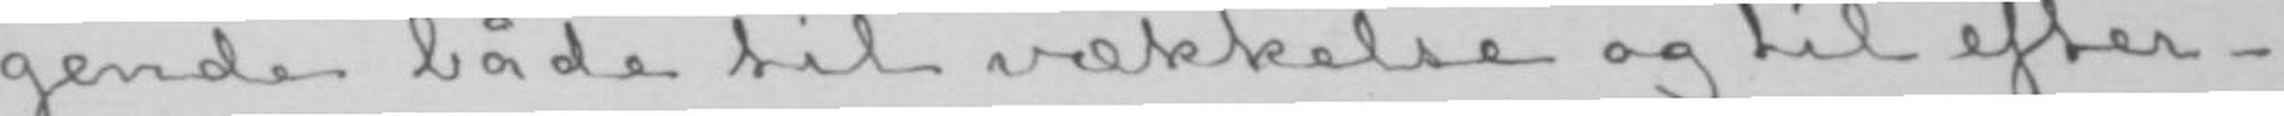gende både til vækkelse og til efter-
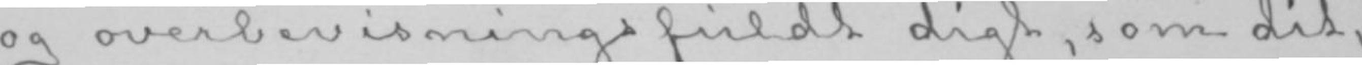og overbevisningsfuldt digt, som dit,
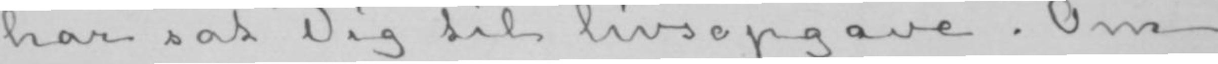har sat Dig til livsopgave. Om
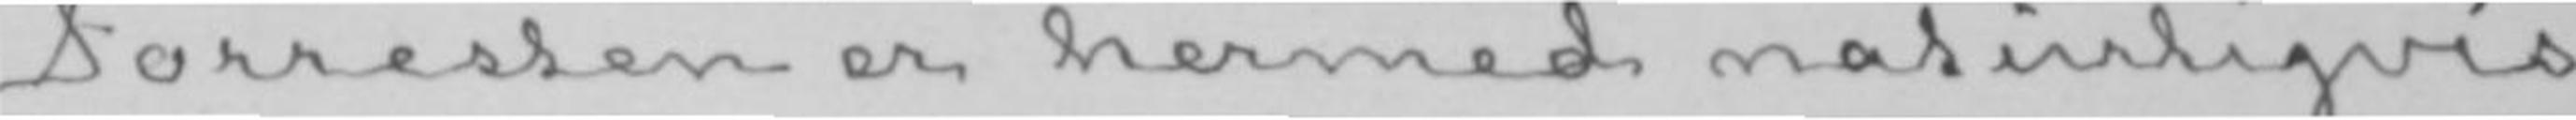Forresten er hermed naturligvis
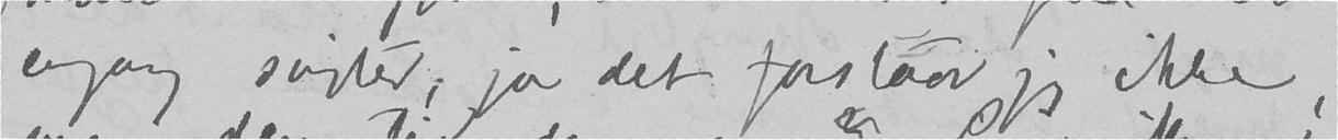engang svigter, ja det forstaar jeg ikke,
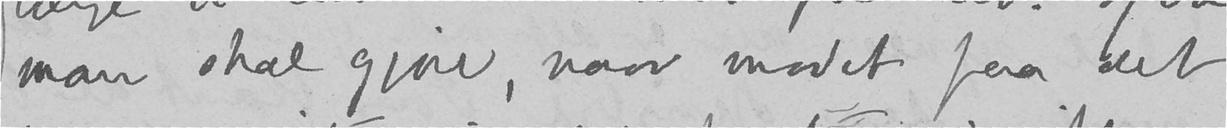man skal gjøre, naar modet paa det
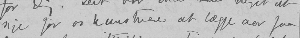sige for os kunstnere at lægge aar paa
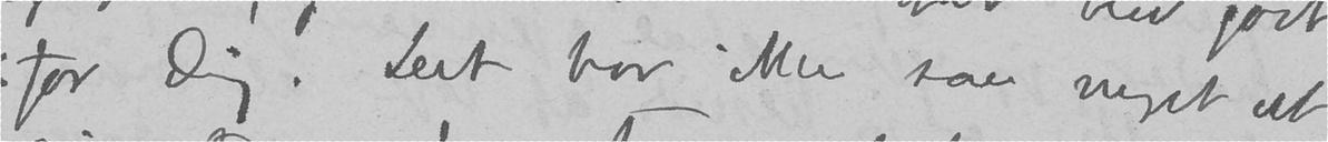for Dig. Det har ikke saa meget at
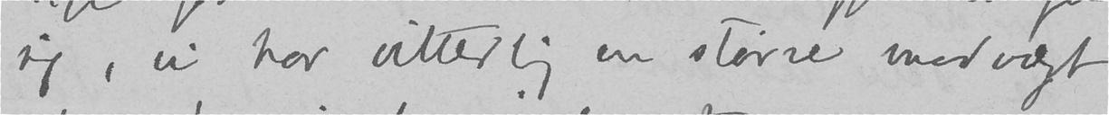sig, vi har vitterlig en større modvægt
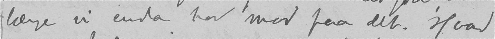længe vi enda tror nok paa det. Hvad
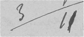3/11
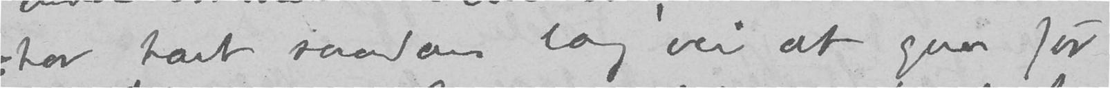har havt saadan lang vei at gaa for
